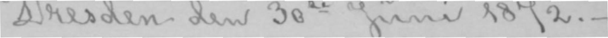Dresden den 30te Juni 1872.-
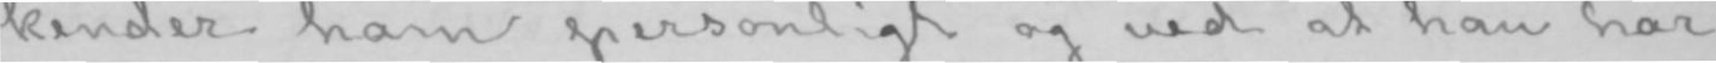kender ham personligt og ved at han har
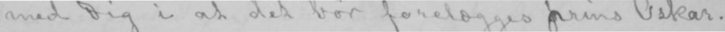med Dig i at det bør forelægges prins Oskar.
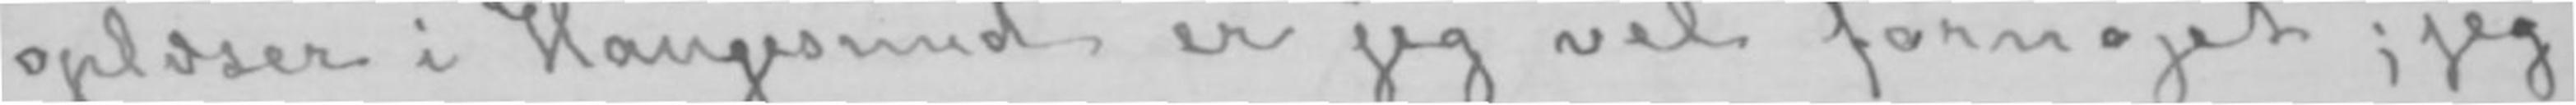oplæser i Haugesund er jeg vel fornøjet; jeg
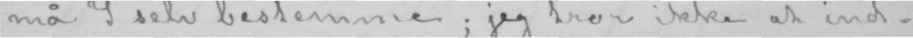må I selv bestemme; jeg tror ikke at ind-
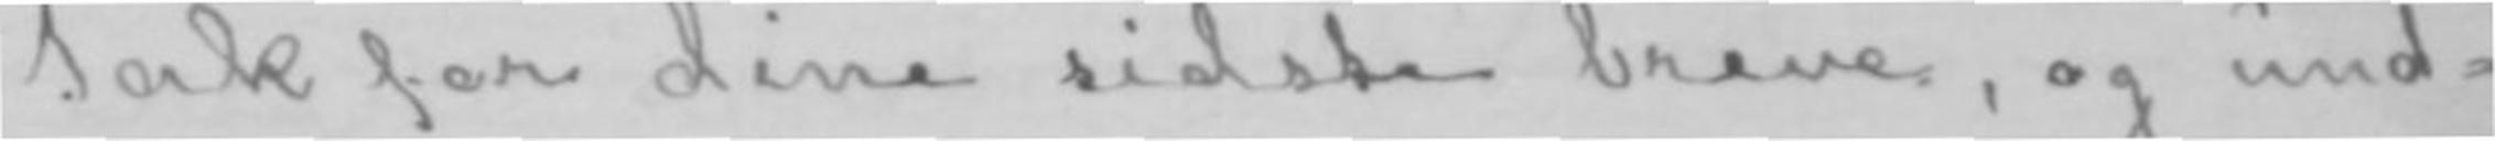Tak for dine sidste breve, og und-
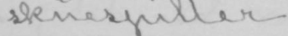skuespiller!
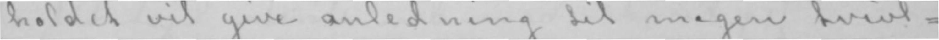holdet vil give anledning til megen tvivl-
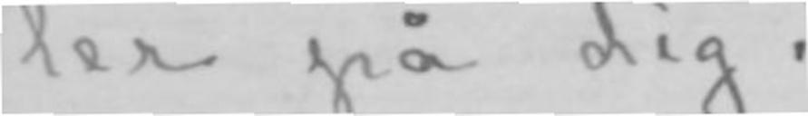ler på dig!
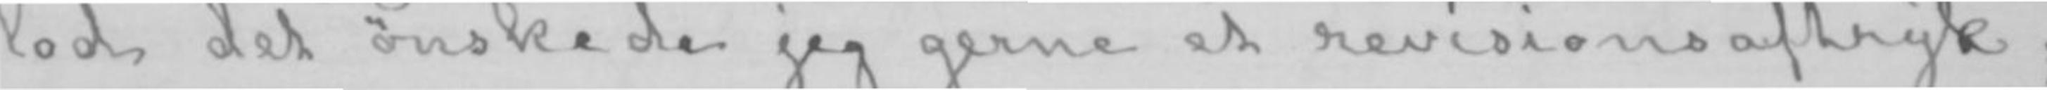lod det ønskede jeg gerne et revisionsaftryk;
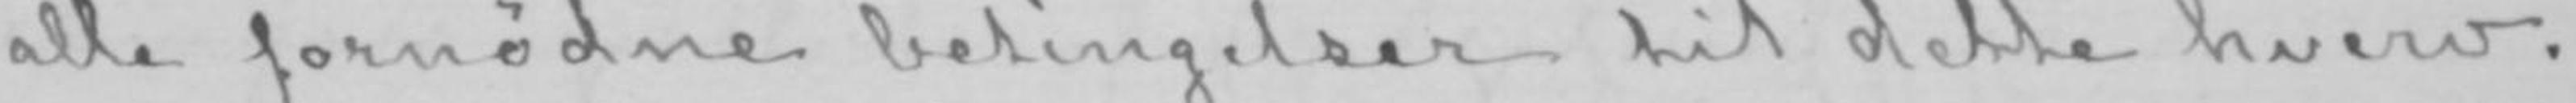alle fornødne betingelser til dette hverv.
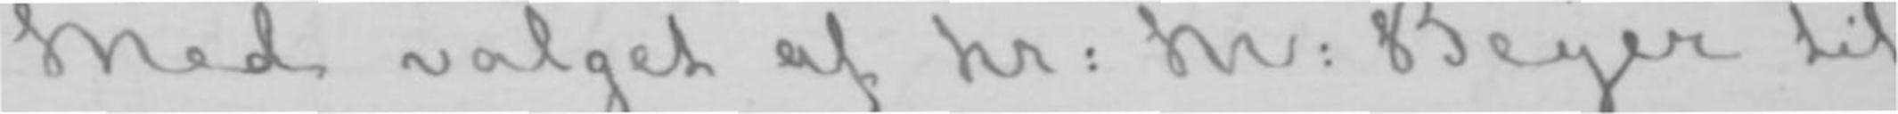Med valget af hr: M: Beyer til
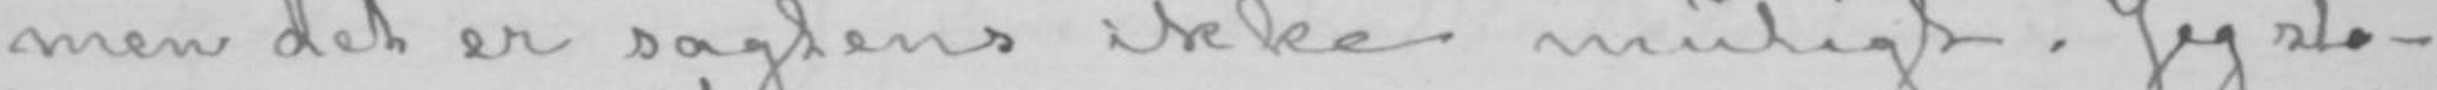men det er sagtens ikke muligt. Jeg sto-
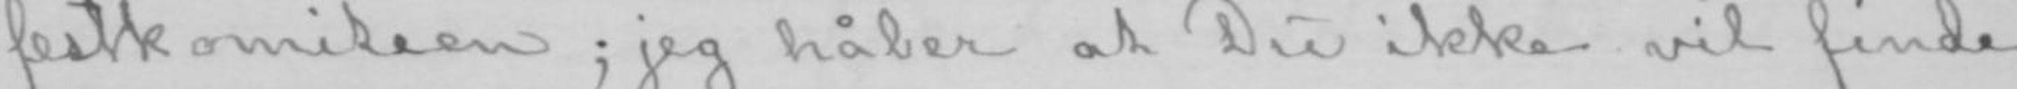festkomiteen; jeg håber at Du ikke vil finde
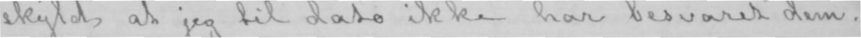skyld at jeg til dato ikke har besvaret dem.
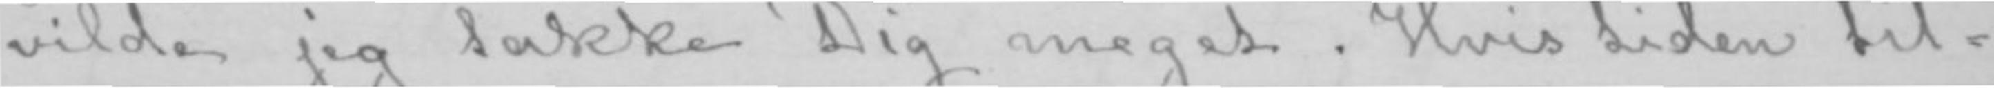vilde jeg takke Dig meget. Hvis tiden til-
